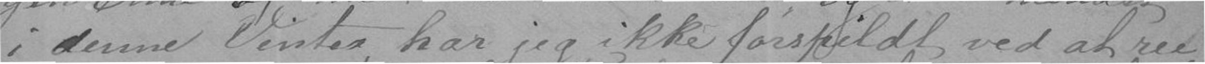i denne Vinter, har jeg ikke forspildt ved at ru-
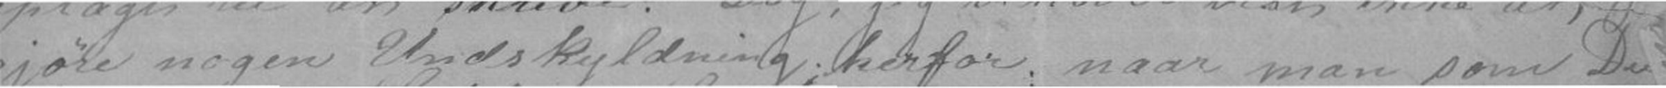gjøre nogen Undskyldning herfor; naar man som Du
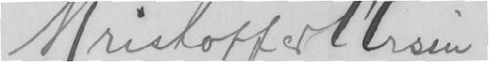Kristoffer Ursin
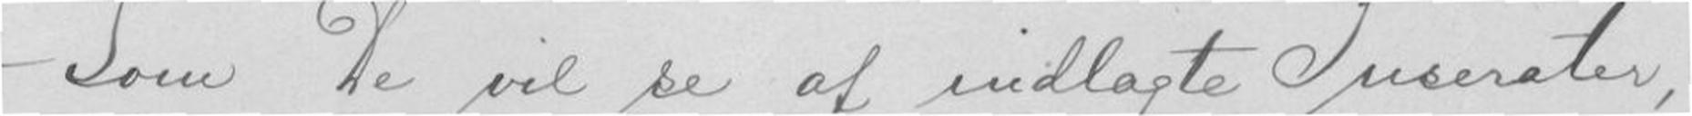Som De vil se af indlagte Inserater,
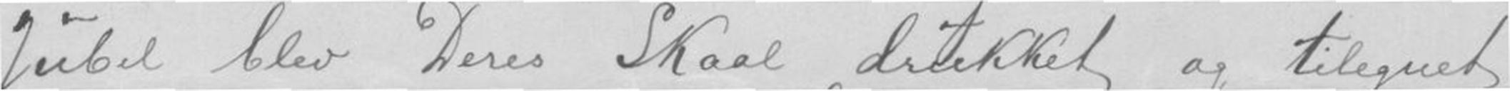Jubel blev Deres Skaal drukket og tilegnet
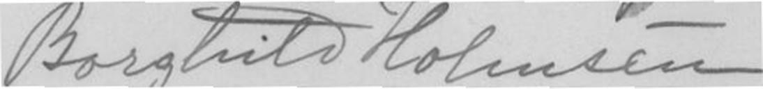Borghild Holmsen
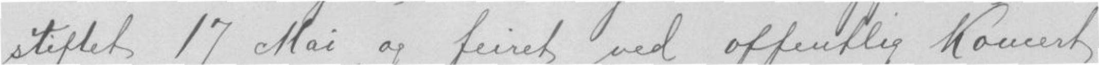stiftet 17 Mai og feiret ved offentlig Koncert
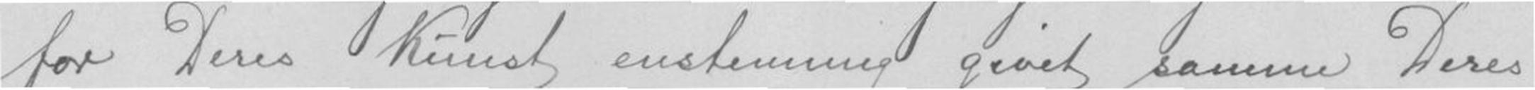for Deres Kunst enstemmig gived samme Deres
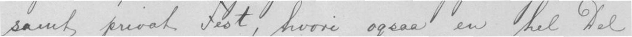samt privat Fest, hvori ogsaa en hel Del
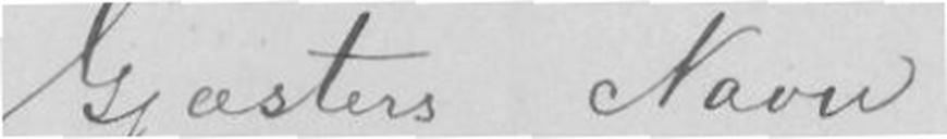Gjæsters Navn
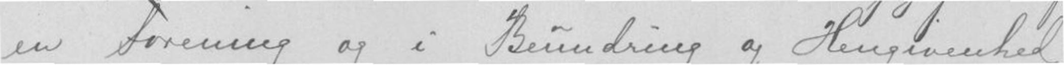en Forening og i Beundring og Hengivenhed
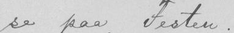se paa Festen.
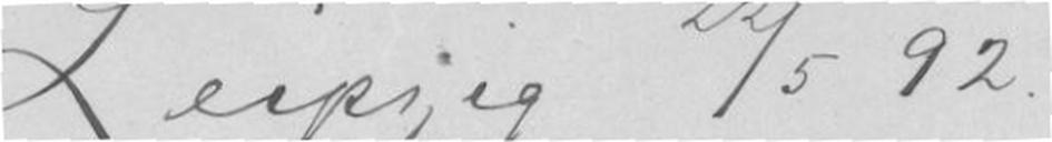Leipzig 22/5-92
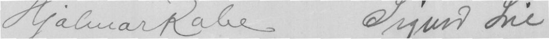Hjalmar Rabe Sigurd Lie
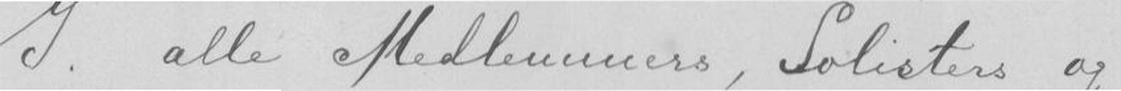I alle Medlemmers, Solister og
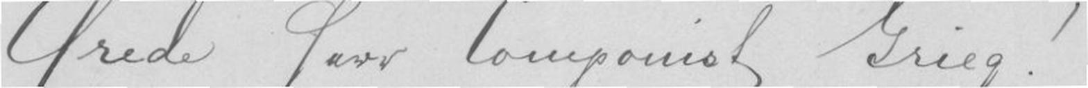Ærede herr Coumponist Grieg!
